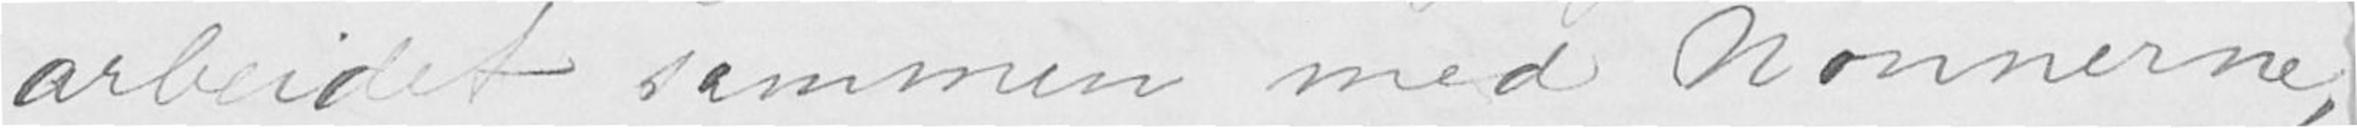arbeidet sammen med nonnerne,
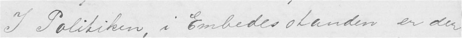I Politiken, i Embedesstanden er der
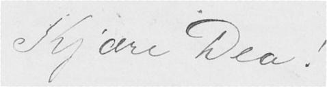Kjære Dea!
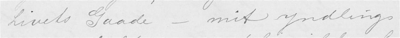Livets Gaade — mit yndlings-
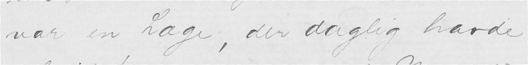var en Læge, der daglig havde
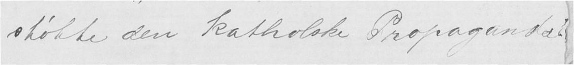støtte den katholske Propaganda".
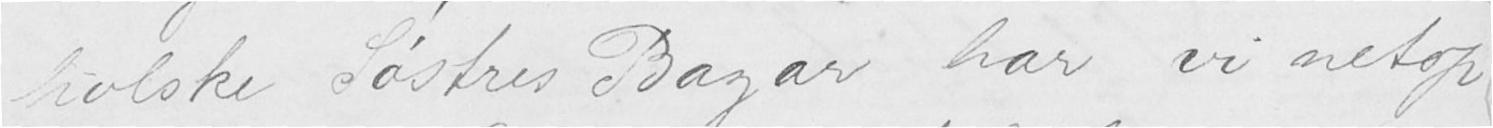holske Søstres Bøger har vi netop
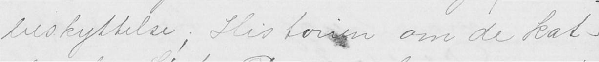beskyttelse; Historen om de kat-
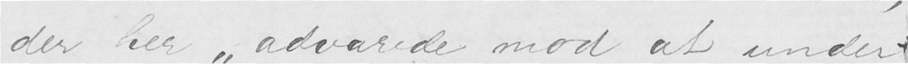der her "advarede mod at under-
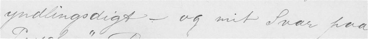yndlingsdigt — og mit Svar paa
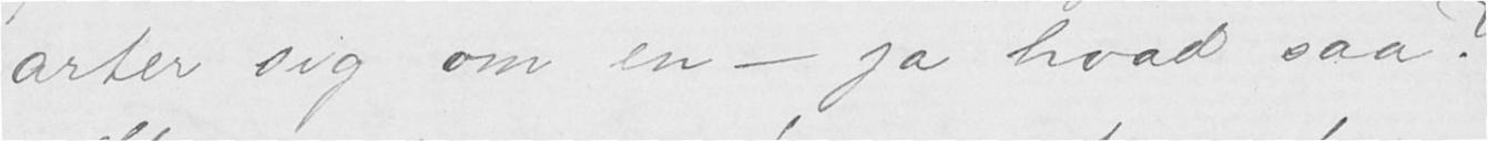arter sig om en — ja hvad saa?
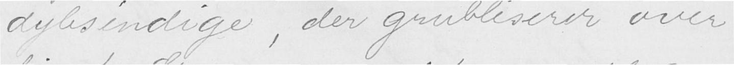dybsindige, der grubliserer over
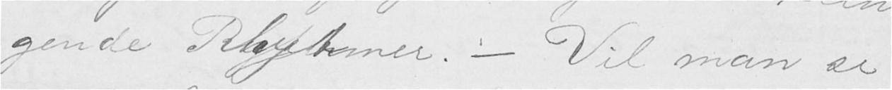gende Rhytmer. — Vil man se
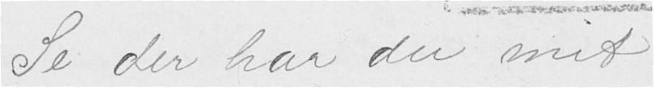Se der har du mit
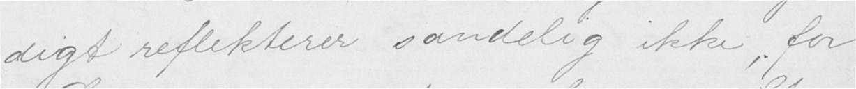digt reflekterer sandelig ikke, for
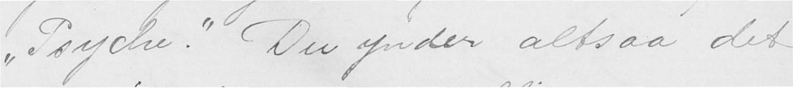"Psyche". Du ynder altsaa det
